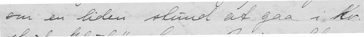om en liden stund at gaa i "Kr.
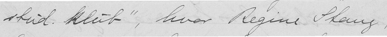stud. klub", hvor Regine Stang,
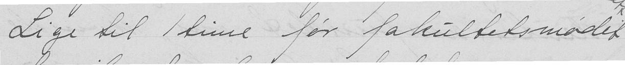Lige til 1 time før fakultetsmødet
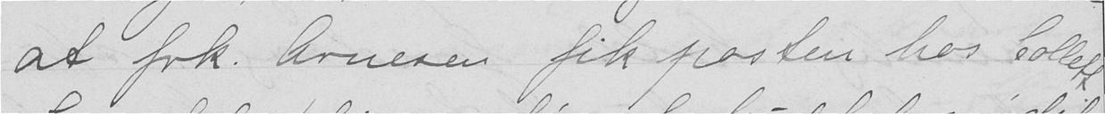at frk. Arnesen fik posten hos Collett.
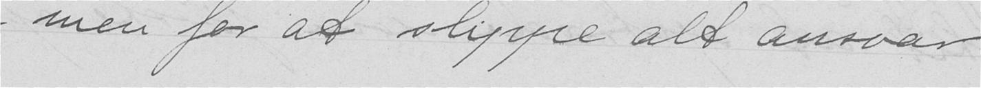- men for at slippe alt ansvar
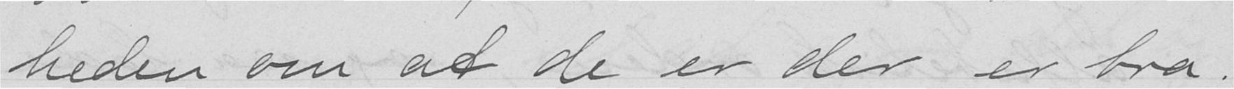heden om at de er der er bra.
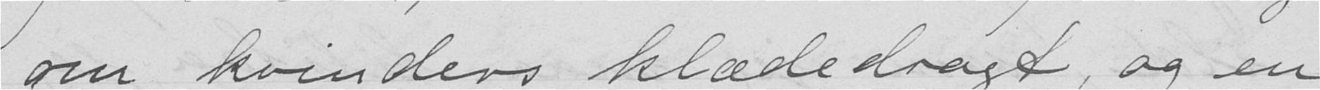om kvinders klædedragt, og en
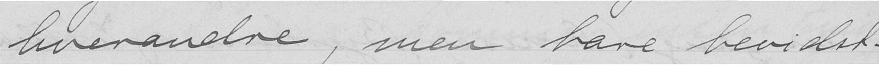hverandre, men bare bevidst-
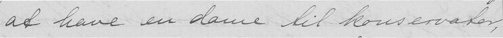at have en dame til konservator,
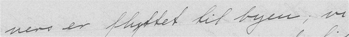ners er flyttet til byen; vi
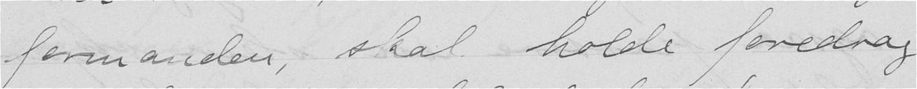formanden, skal holde foredrag
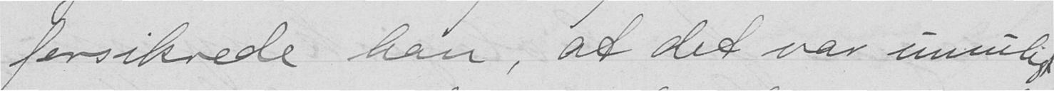forsikrede han, at det var umuligt
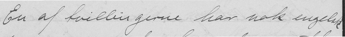En af tvillingerne har nok engelsk
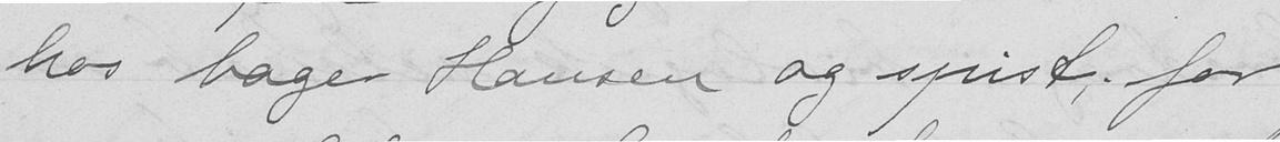hos bager Hansen og spist; for
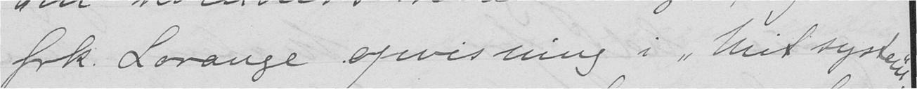frk. Lorange opvisning i "Mit system".
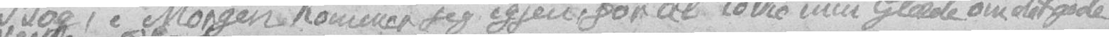Bog, i Morgen kommer jeg igjen, for at tolke min Glæde om det gode
